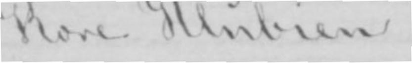Kære Klubien!
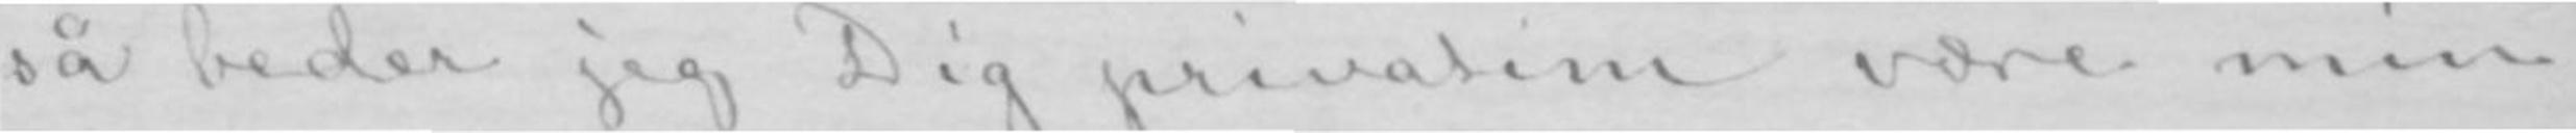så beder jeg Dig privatim være min
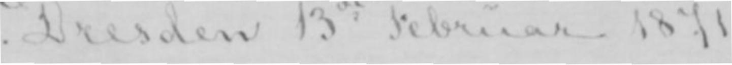Dresden 13de Februar 1871.-
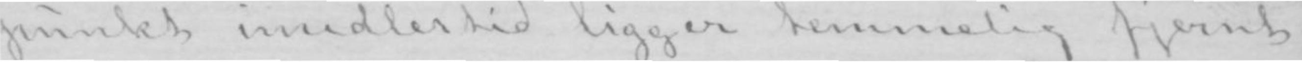punkt imidlertid ligger temmelig fjernt,
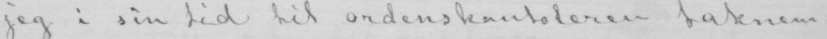jeg i sin tid til ordenskantsleren taknem-
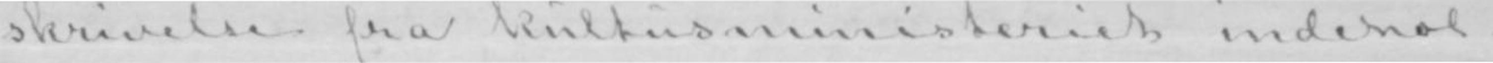skrivelse fra kultusministeriet indehol-
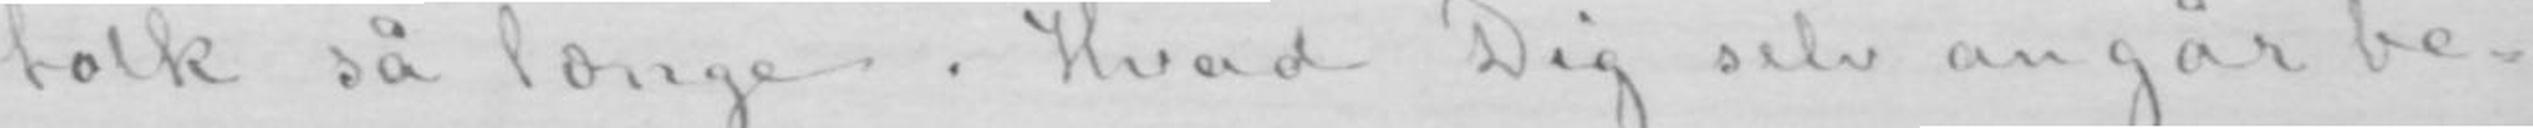tolk så længe. Hvad Dig selv angår be-
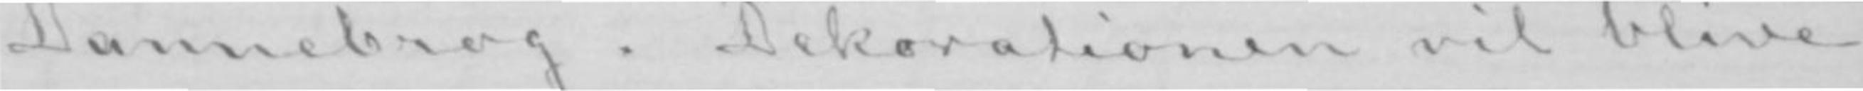Dannebrog. Dekorationen vil blive
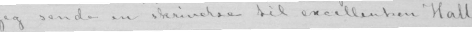jeg sende en skrivelse til excellentsen Hall
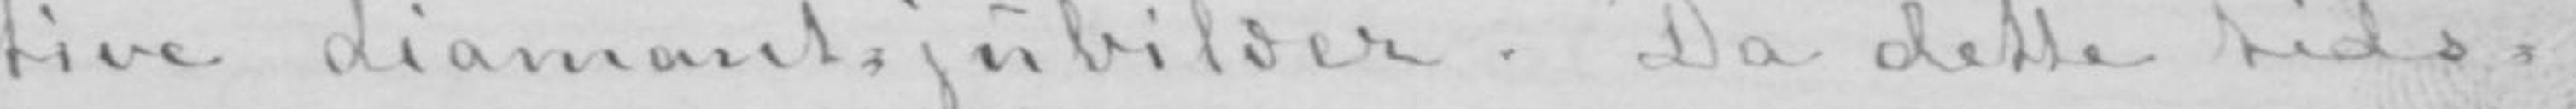tive diamant-jubilæer. Da dette tids-
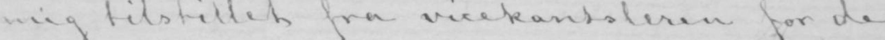mig tilstillet fra vicekantsleren for de
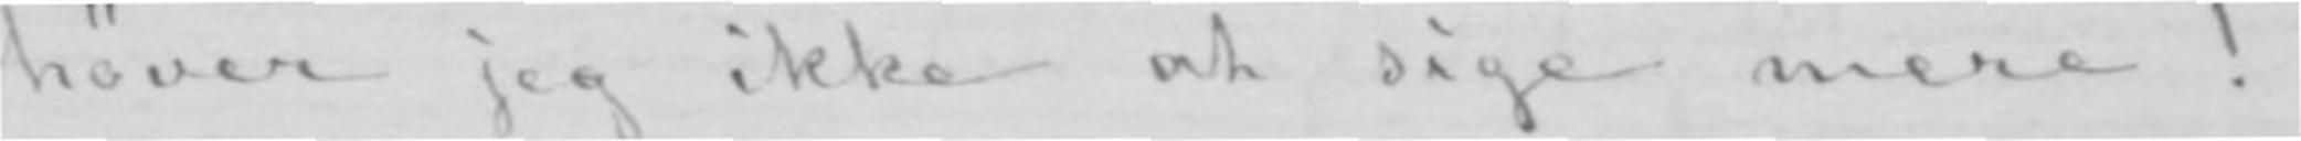høver jeg ikke at sige mere!
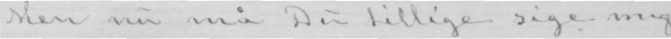Men nu må Du tillige sige mig
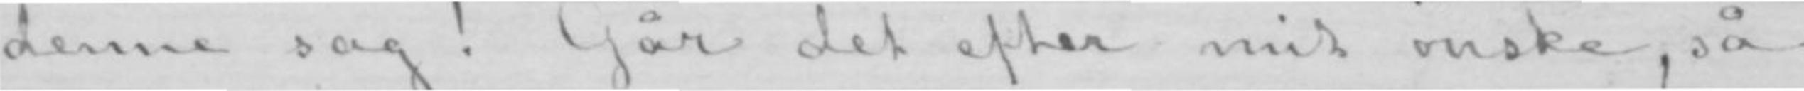denne sag! Går det efter mit ønske, så
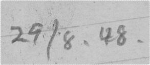29/8. 48.
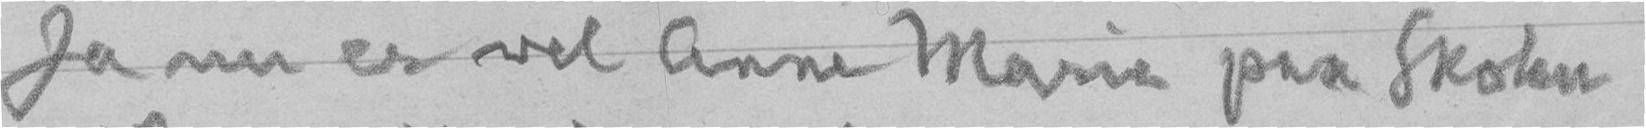Ja nu er vel Anne Marie paa Skolen
